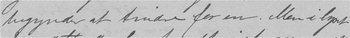begynder at tindre for en. Men i lyset
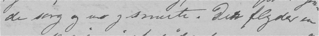de sorg og uro og smerte. Det flyder en
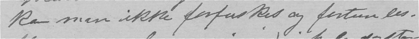kan man ikke forfuskes og fortumles.
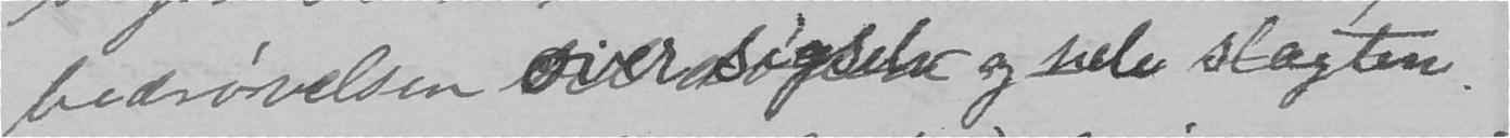bedrøvelsen over sig selv og hele slægten.
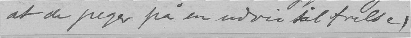at de peger på en udvei til frelse,
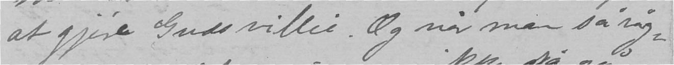at gjøre Guds villie. Og når man så våg-
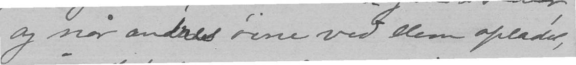og når andres øine ved dem oplades,
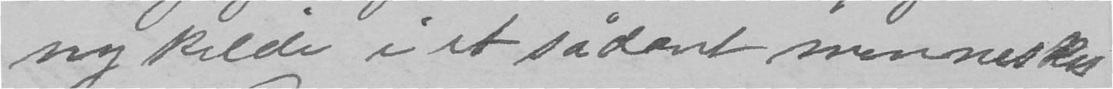ny kilde i et sådant menneskes
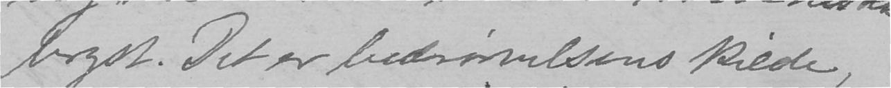bryst. Det er bedrøvelsens kilde,
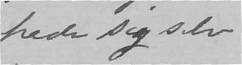hade sig selv
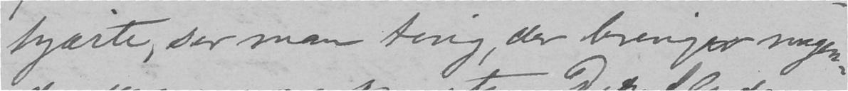hjærte, ser man ting, der bringer snigen-
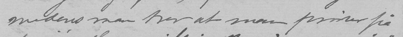medens man tror at man prøver på
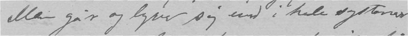Man går og lyver sig ind i hele systemer
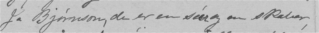Ja Bjørnson, de er en séer og skaber,
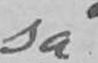så
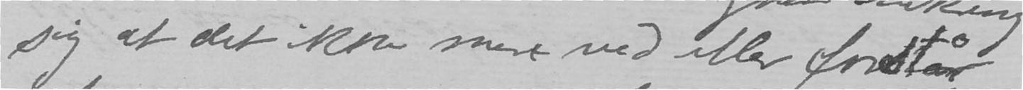sig at det ikke mere ved eller forstår
